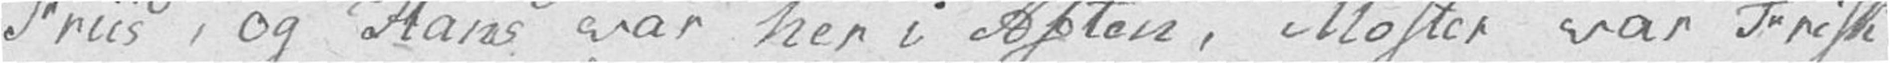Friis, og Hans var her i Aften, Moster var Frisk
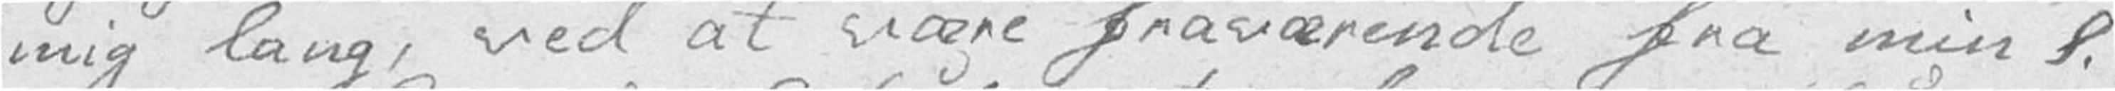mig lang, ved at være fraværende fra min S.
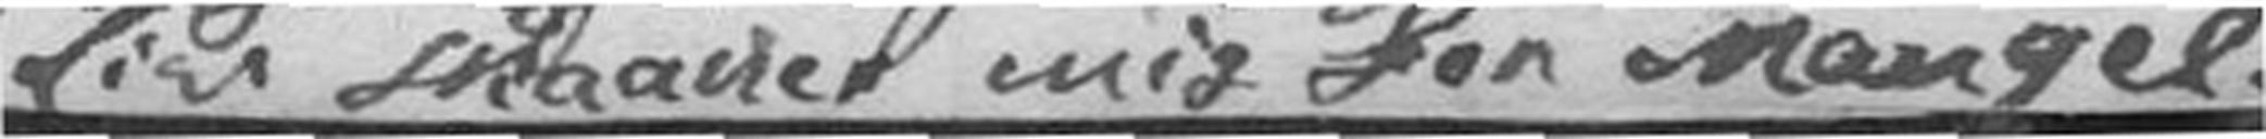Liv skaaner mig for Mangel.
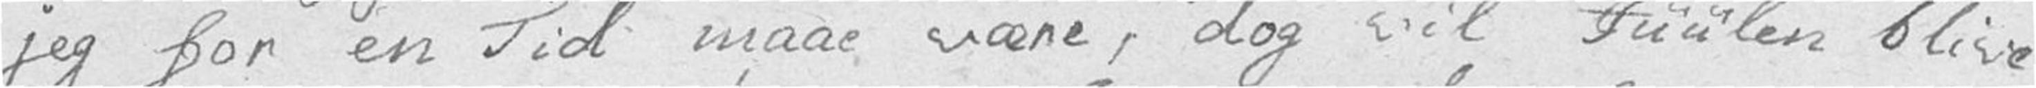jeg for en Tid maae være, dog vil Juulen blive
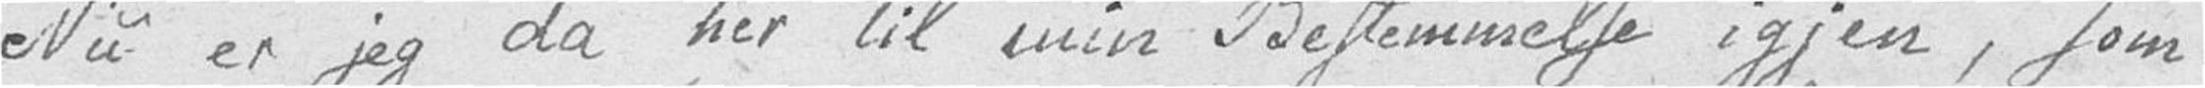Nu er jeg da her til min Bestemmelse igjen, som
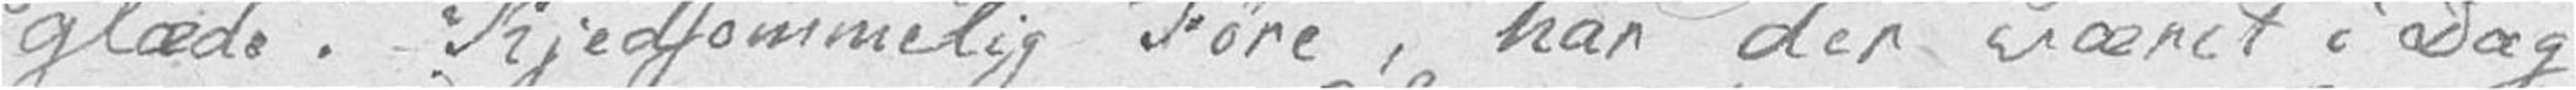glæde. Kjedsommelig Føre, har der været i Dag
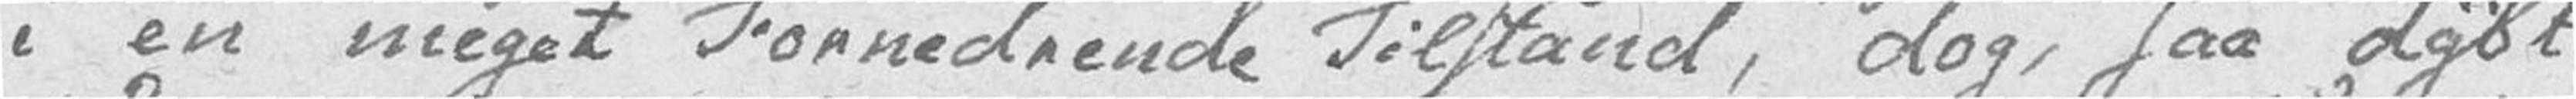i en meget Fornedrende Tilstand, dog, saa dybt
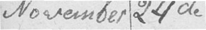November 24de
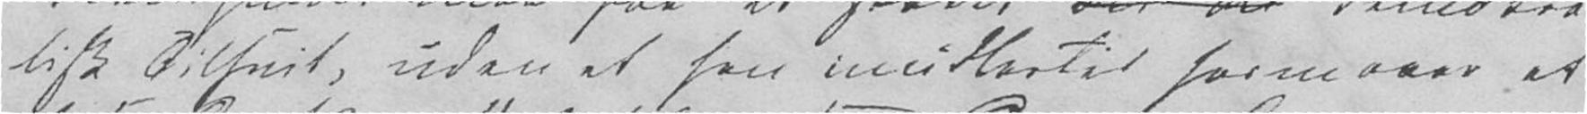tisk Tilsnit, uden at han imidlertid formaaer at
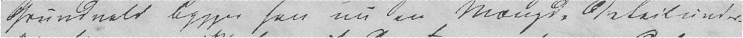Grundvold bygger han nu en Mængde Detailunder-
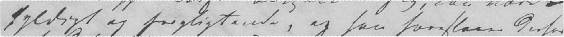gyldigt og forpligtende, og han foreslaaer derfor
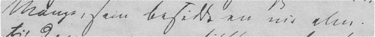Mange, som besidde en vis alm.
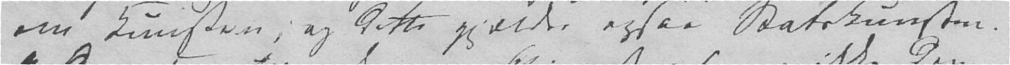om Kunsten, og dette gjælder ogsaa Statskunsten.
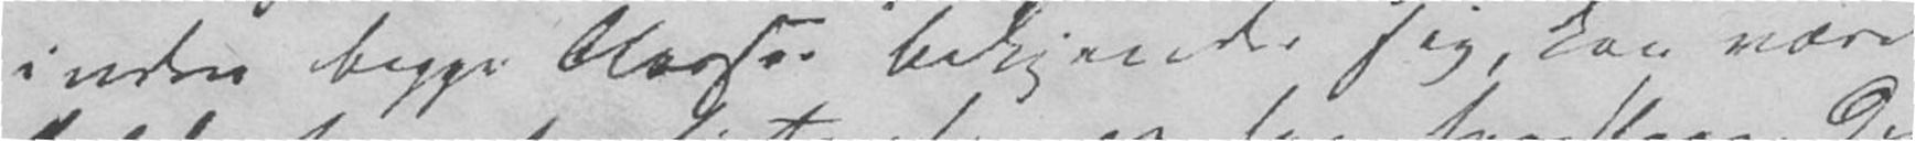inden begge Classer bekjender sig, kan være
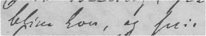blive Lov, og hvis
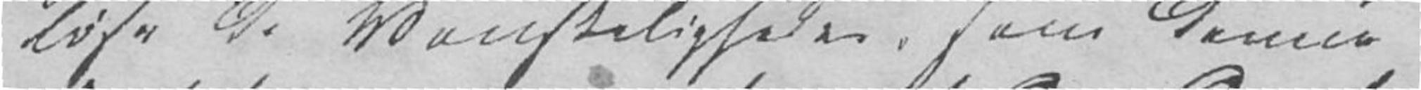løse de Vanskeligheder, som denne
